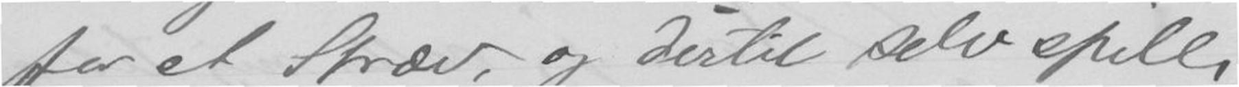for et Stræv, og dertil selv spille
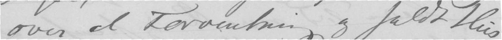over al Forventning og fuldt Hus
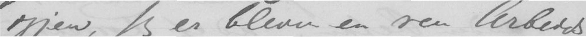igjen, jeg er bleven en ren Ardeidshest,
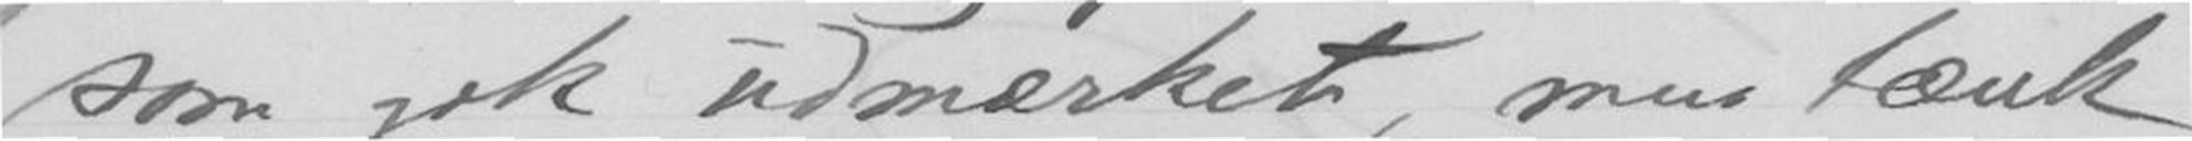som gik udmærket, men tænk
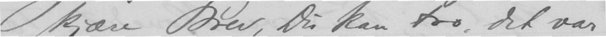kjære Brev, Du kan tro det var
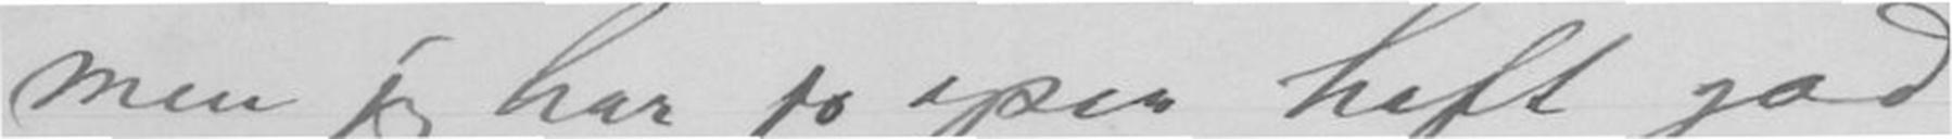men jeg har jo ogsaa haft god
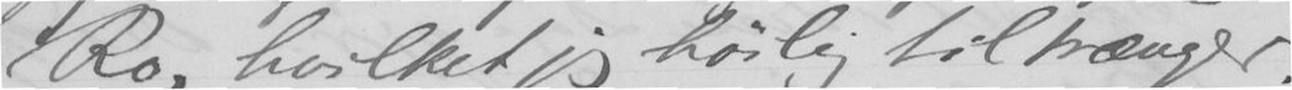Ro, hvilket jeg høilig tiltrænger.
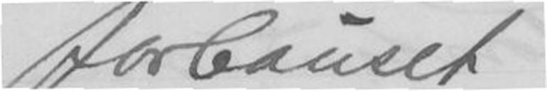forbauset
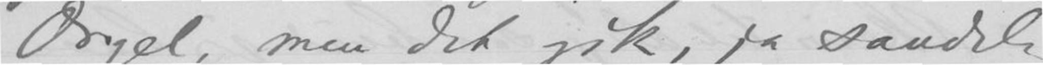Orgel, men det gik, jo sandelig
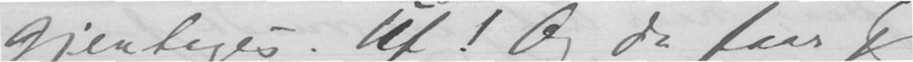gjentages. Uf! Og da faar jeg
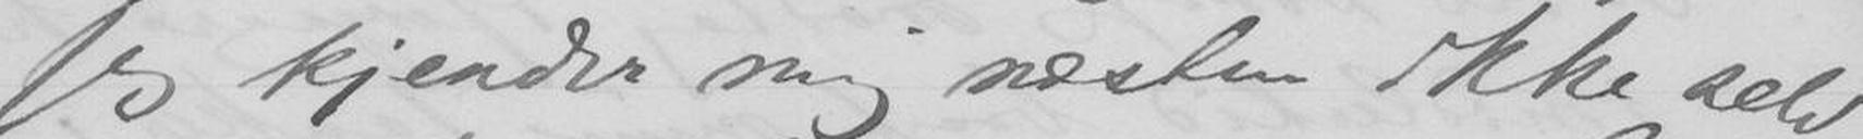Jeg kjender mig næsten ikke selv
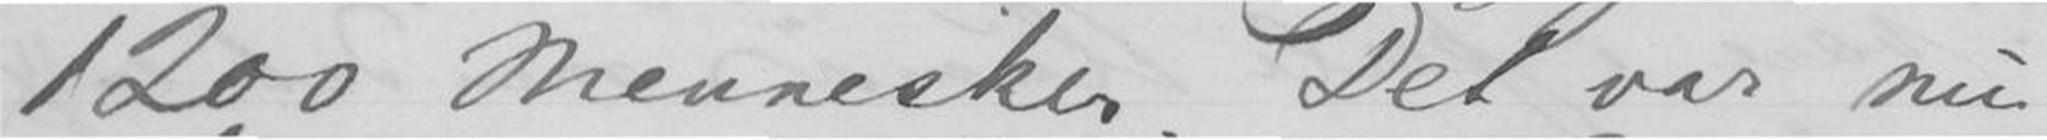1200 Mennesker. Det var nu det.
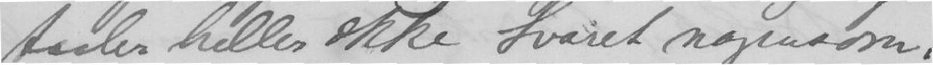taales heller ikke Svaret nogensom,
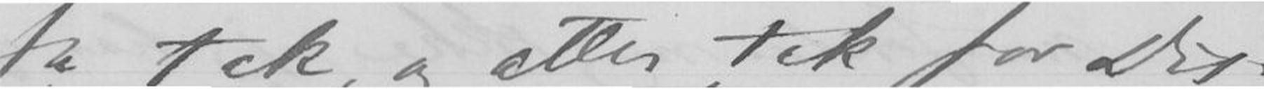Ja tak og atter tak for Dit
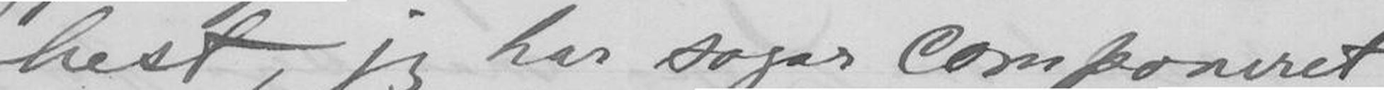jeg har sogar Componeret
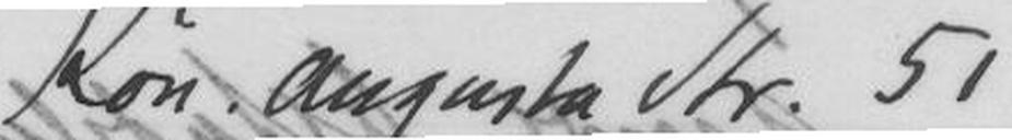Kön. Augusta Str. 51
